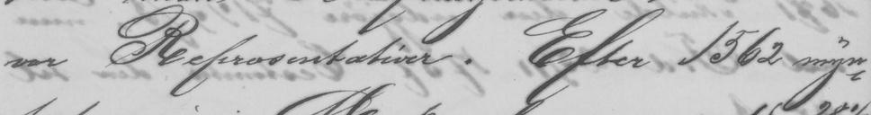var Representativer. Efter 1562 myn-
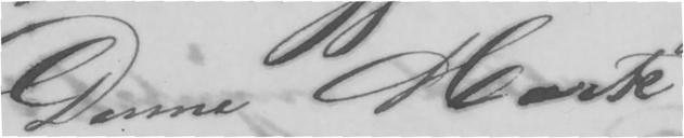Denne Mark
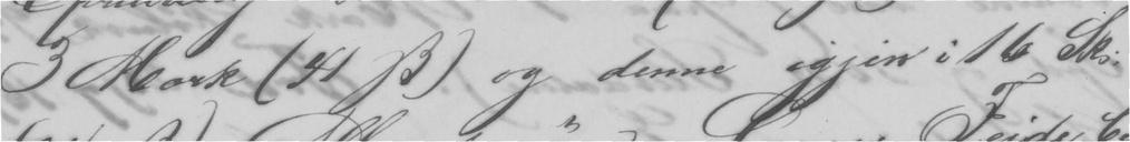3 Mark (41 ẞ) og denne igjen i 16 Sk:
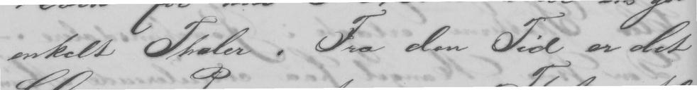enkelt Thaler. Fra den Tid er det
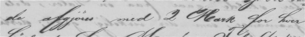de afgjøres med 2 Mark for hver
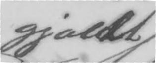gjaldt
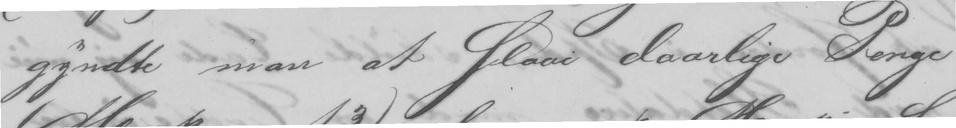gyndte man at Slaa daarlige Penge
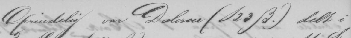Opridelig var Dalerne (123 ẞ.) delt i
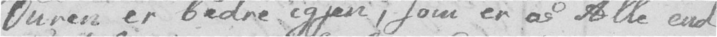Ouren er bedre igjen, som er os Alle end
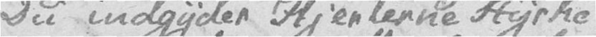Du indgyder Hjerterne Styrke
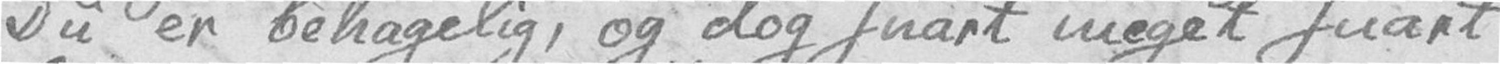Du er behagelig, og dog snart meget snart
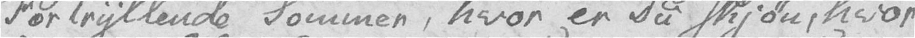Fortryllende Sommer, hvor er Du skjøn, hvor
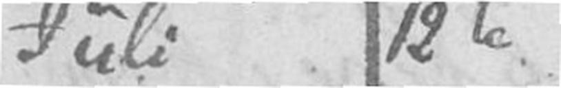Juli 12te
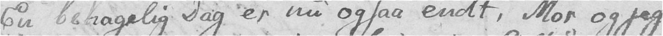En behagelig Dag er nu ogsaa endt, Mor og jeg
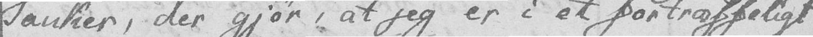Tanker, der gjør, at jeg er i et fortræffeligt
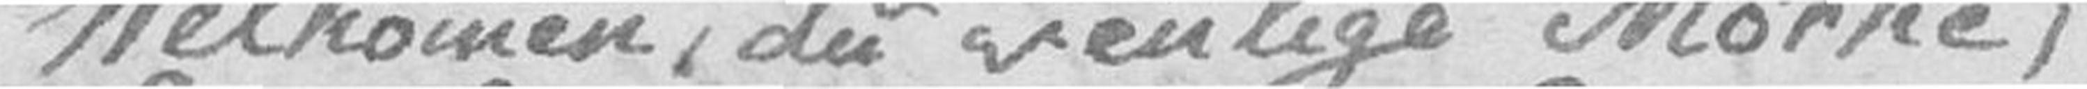Velkommen, du venlige Mørke,
